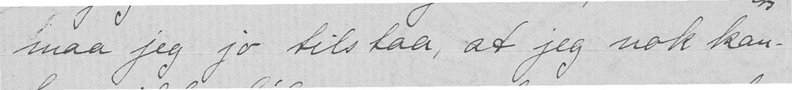maa jeg jo tilstaa, at jeg nok kan-
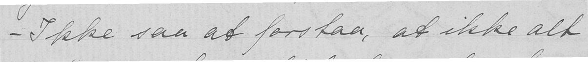- Ikke saa at forstaa, at ikke alt
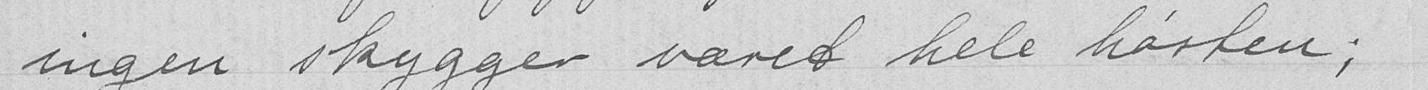ingen skygger været hele høsten;
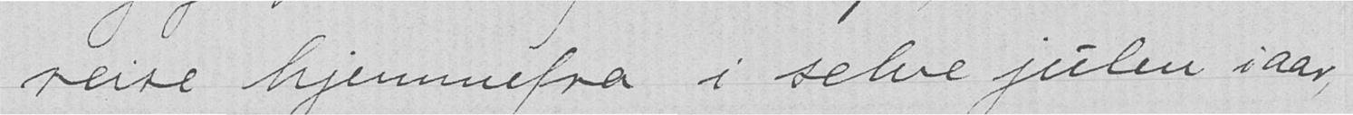reise hjemmefra i selve julen iaar,
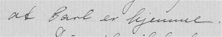at Carl er hjemme.
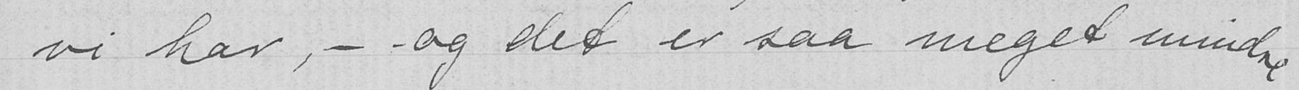vi har, - - og det er saa meget mindre
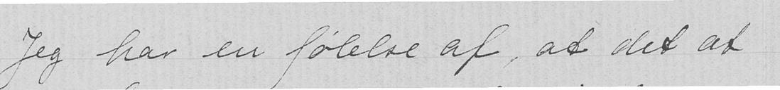Jeg har en følelse af, at det at
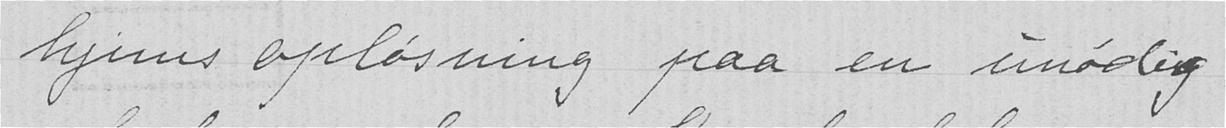hjems opløsning paa en unødig
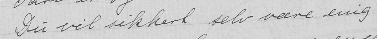Du vil sikkert selv være enig
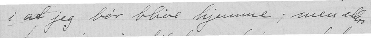i at jeg bør blive hjemme; men ellers
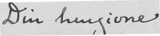Din hengivne
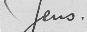Jens.
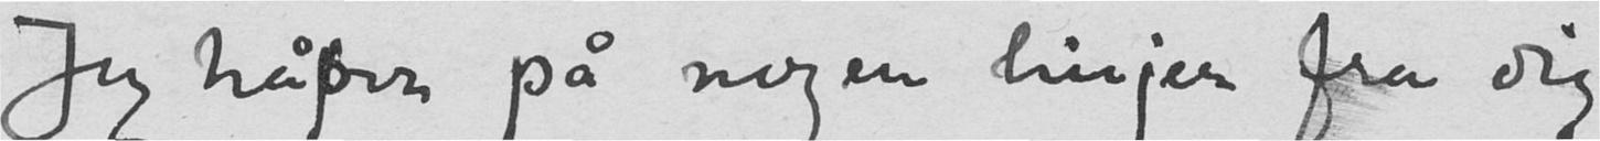Jeg håper på nogen linjer fra dig
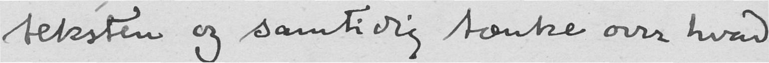teksten og samtidig tænke over hvad
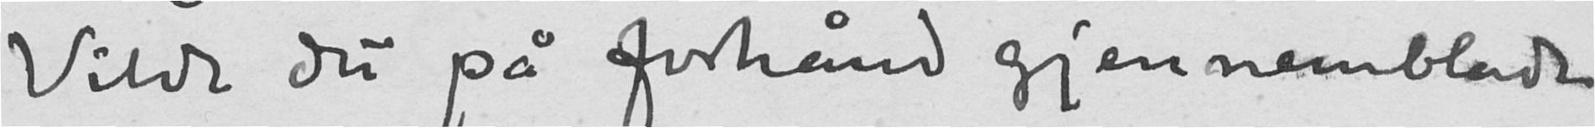Vilde du på forhånd gjennemblade
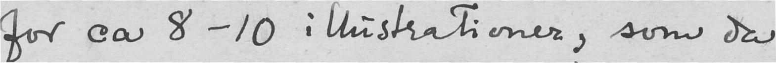for ca 8-10 illustrationer, som da
